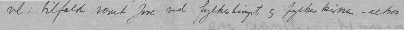vel i tilfælde været fore ved fylkestinget og fylkeskirken – altsaa
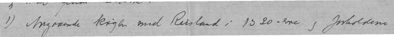1) Angaaende krigen med Rusland i 1320-erne og forholdene
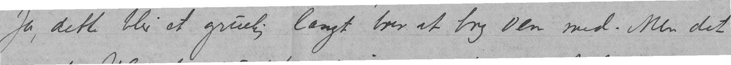Ja, dette blir et gruelig langt brev at bry Dem med. Men det
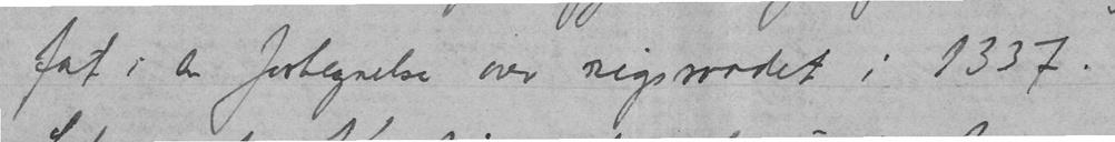fat i en fortegnelse over rigsraadet i 1337.
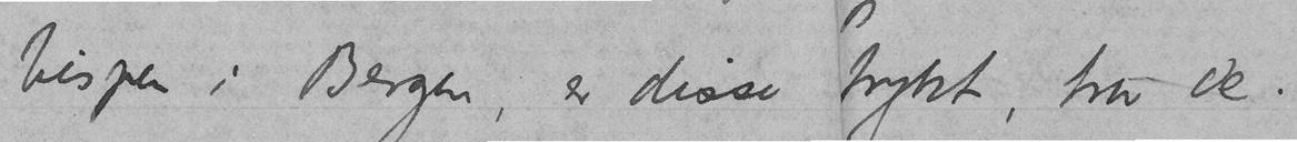bispen i Bergen, er disse trykt, tror De.
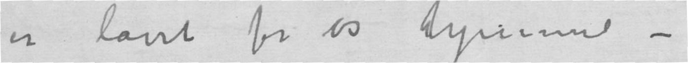erlavet for os hjemme –
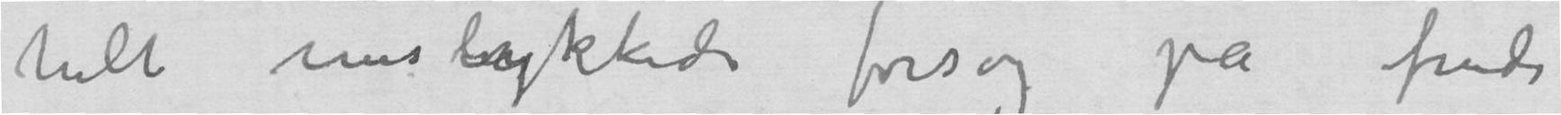helt mislykkede forsøg på finde
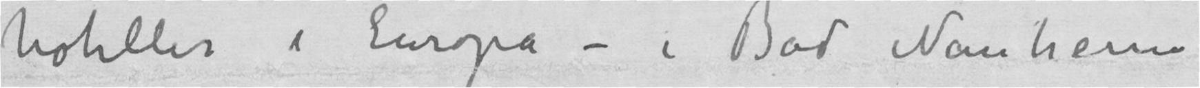hoteller i Europa – i Bad Nauheim
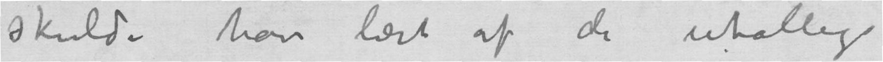skulde have lært af de utallige
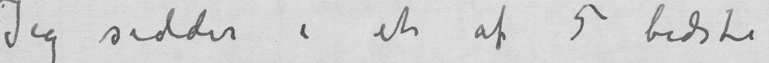Jeg sidder i et af 5 bedste
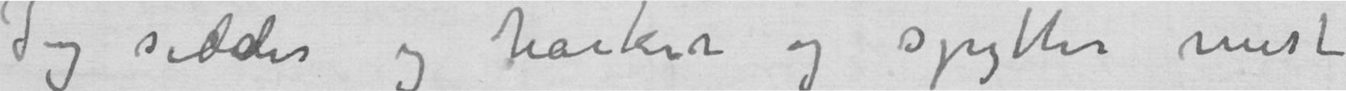Jeg sidder og harker og spytter mest
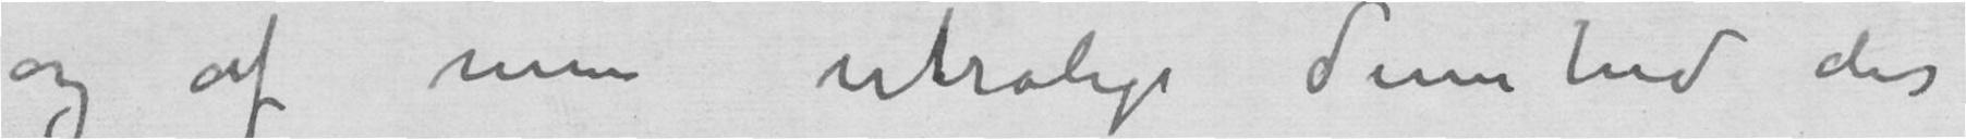og af min utrolige dumhed der
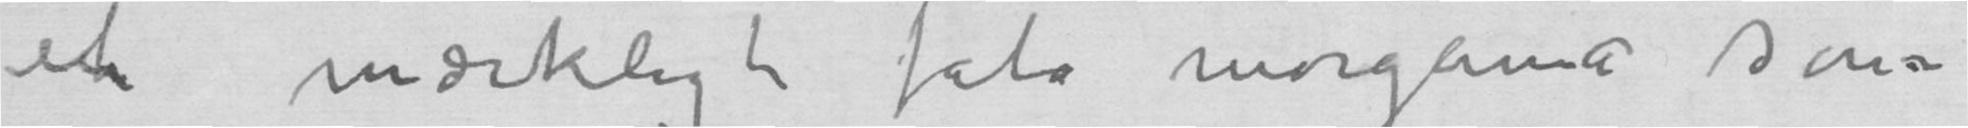et mærkligt fata morgana som
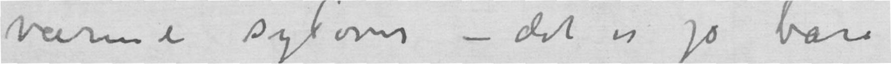varme sydover – det er jo bare
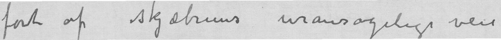ført af skjæbnens uransagelige veie
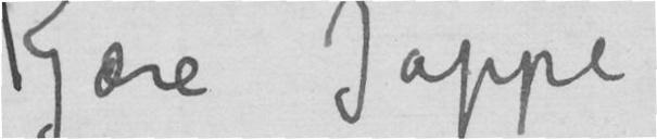Kjære Jappe
